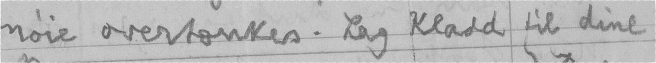nøie overtænkes. Lag Kladd til dine
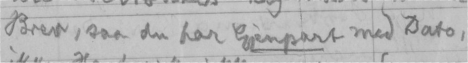Brev, saa du har Gjenpart med Dato,
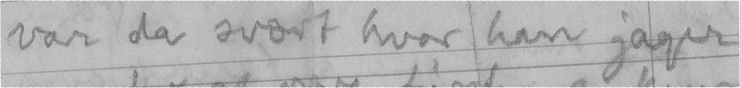var da svært hvor han jager
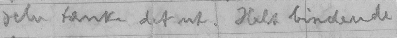selv tænke det ut. Helt bindende
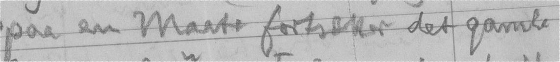paa en Maate fortsætter det gamle
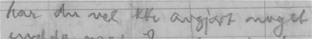har du vel ikke avgjort noget
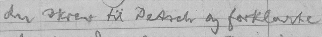du skrev til Detsch og forklarte
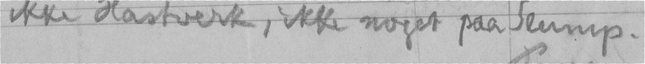ikke Hastverk, ikke noget paa Slump.
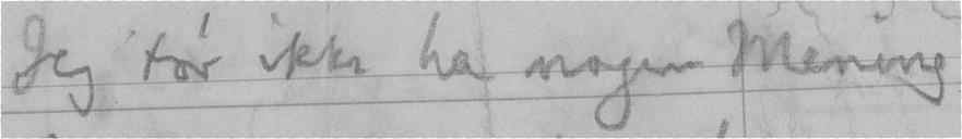Jeg tør ikke ha nogen Mening
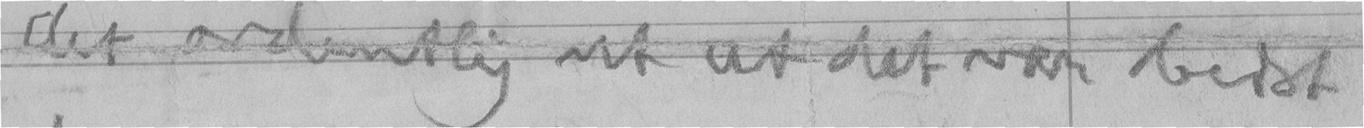det ordentlig ut at det var bedst
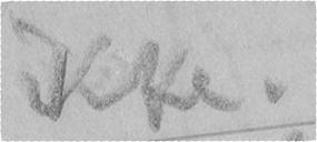ikke.
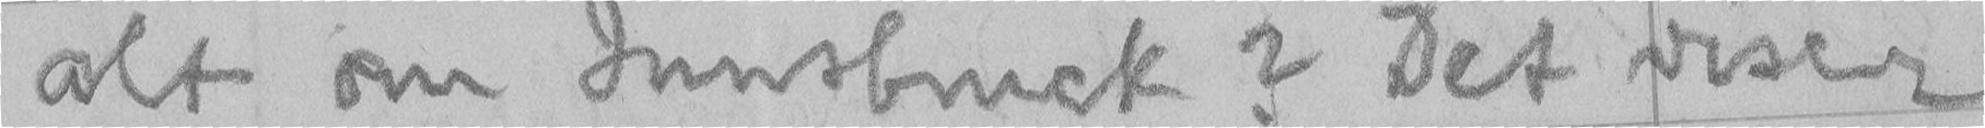alt om Innsbruck? Det viser
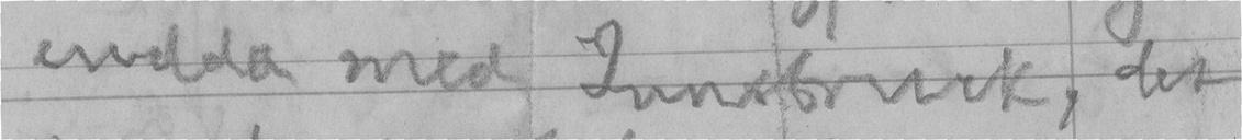endda med Innsbruck, det
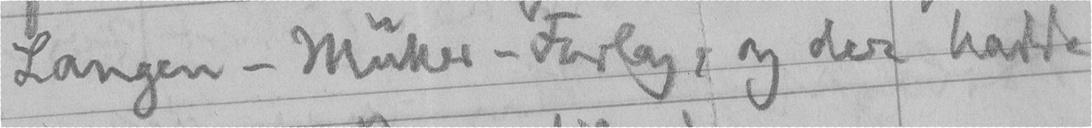Langen-Müller-Forlag, og der hadde
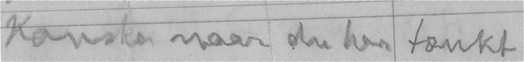Kanske naar du har tænkt
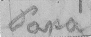Papa
